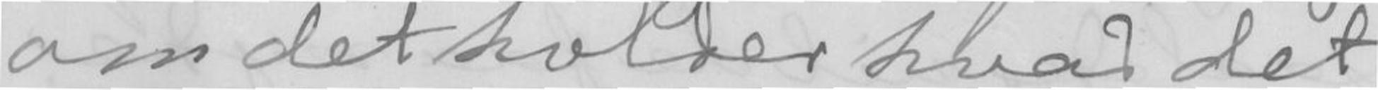om det holder hvad det
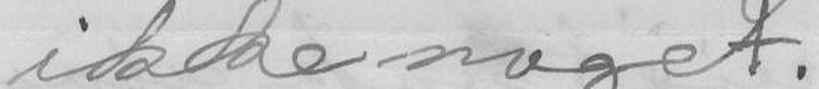ikke noget.
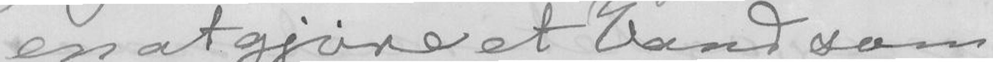en at gjøre et Vand som
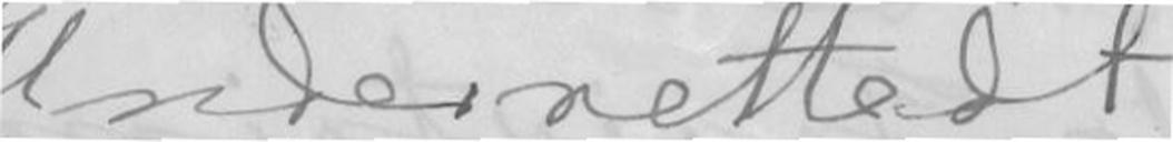Underrettedt
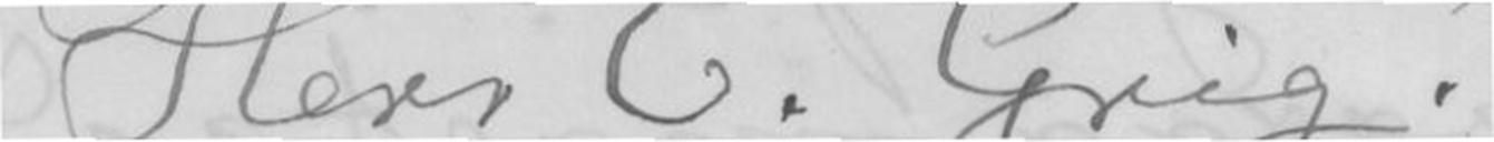Herr E. Grieg!
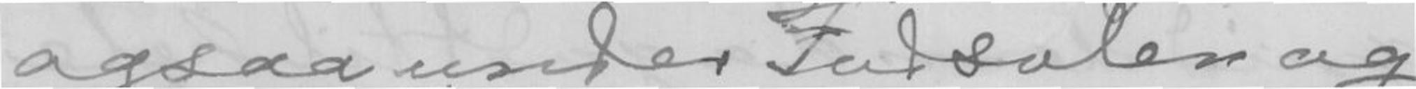ogsaa under Fotsolen og
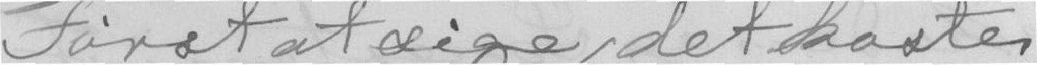Først at sige, det koster
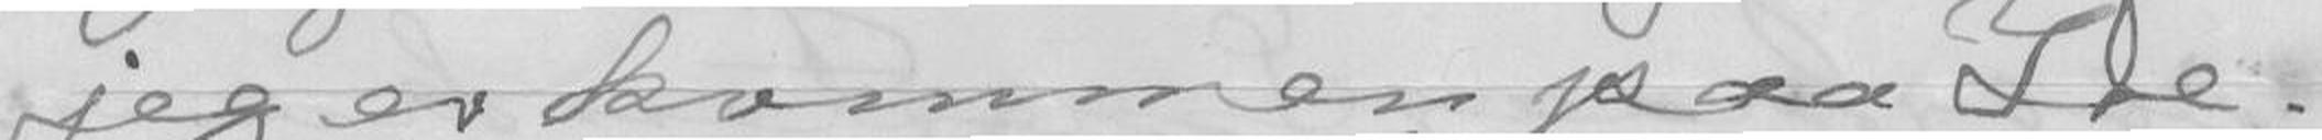jeg er kommen paa Ide-
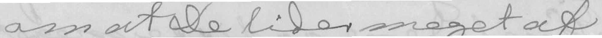om at De lider meget af
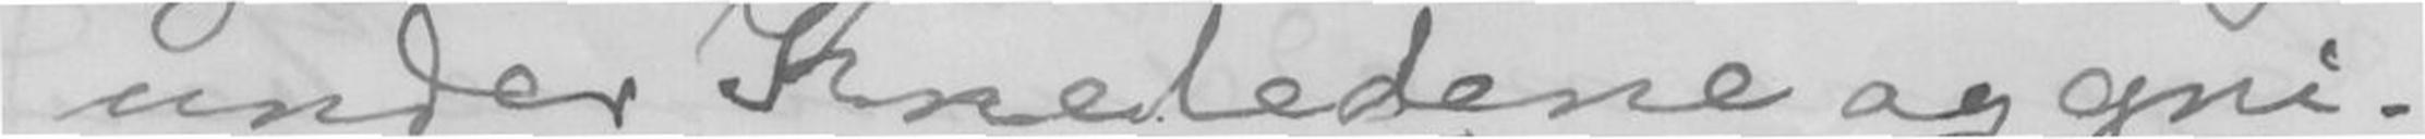under Kneledene og gni-
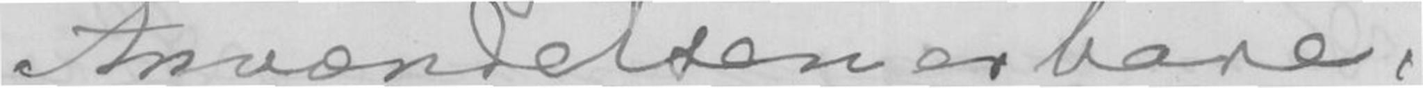Anvændelsen er bare,
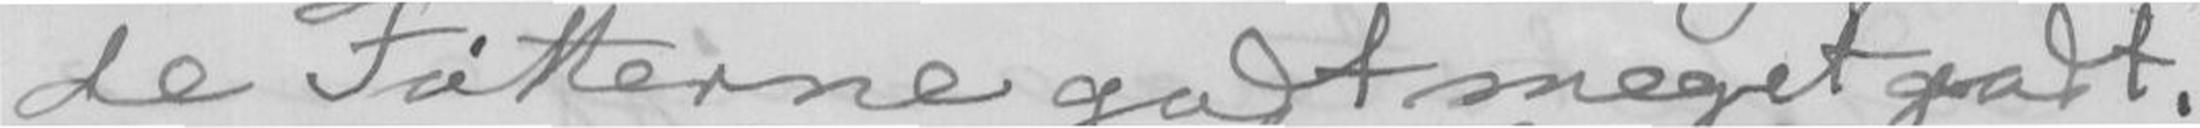de Føtterne godt meget godt.
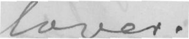lover.
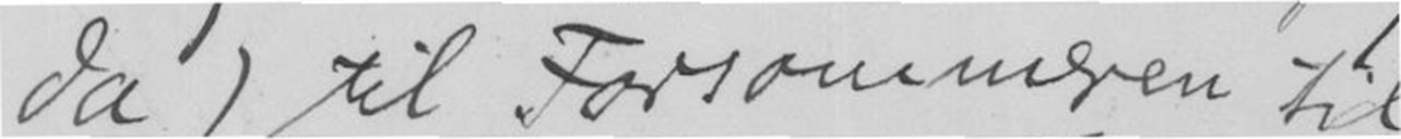da) til Forsommeren til
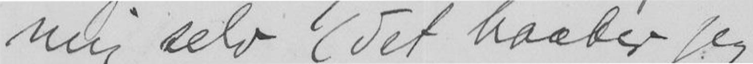mig selv (det haaber jeg
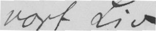vort Liv!
